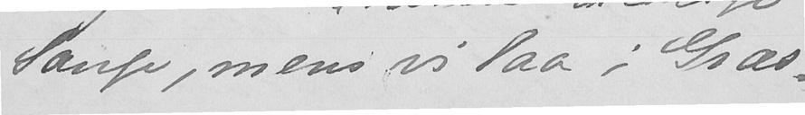Sange, mens vi laa i Græs-
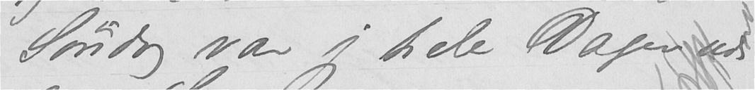Søndag var jeg hele Dagen ud
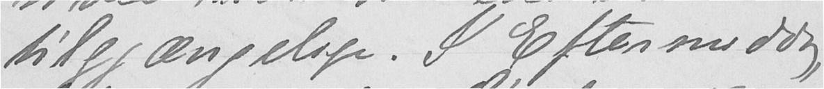tilgjængelige. I Eftermiddag,
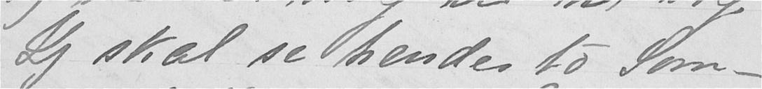Jeg skal se hendes to Som-
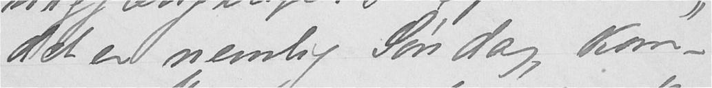det er nemlig Søndag, kom-
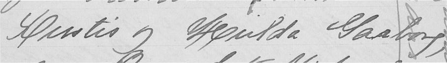Rustis og Hulda Garborg,
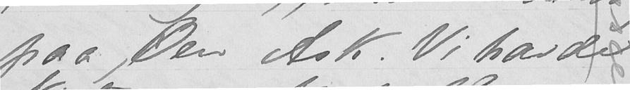paa Øen Ask. Vi havde
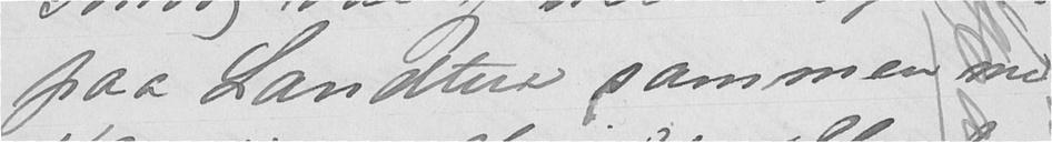paa Landtur sammen med
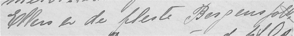Ellers er de fleste Bergensfolk
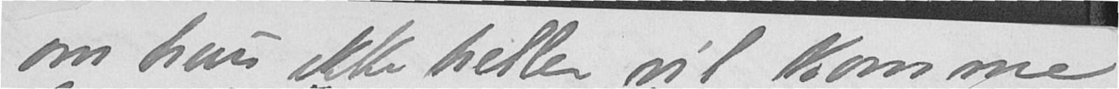om hun ikke heller vil komme
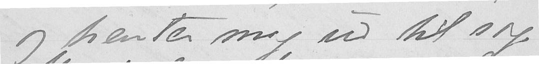og henter mig ud til sig.
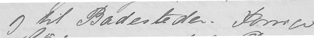og til Badesteder. Forrige
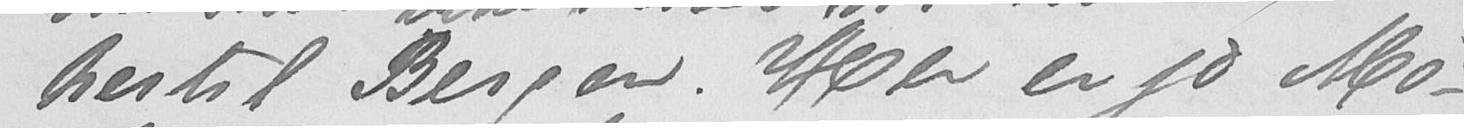hertil Bergen. Her er jo Mo-
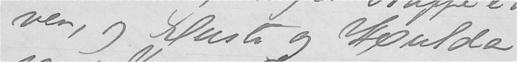ven, og Rusti og Hulda
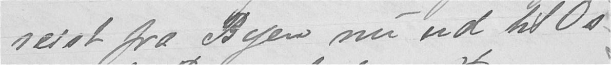reist fra Byen nu ud til Os
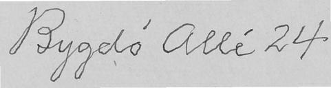Bygdø Allé 24
